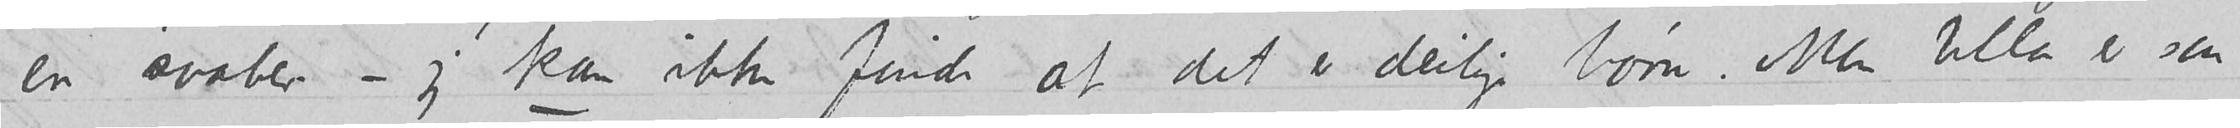en svaber  – jeg kan ikke finde at det er deilige børn.  Men Ulla er saa
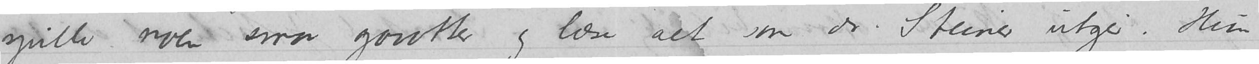spille noen smaa gavotter og læse alt som dr. Steiner utgir. Hun
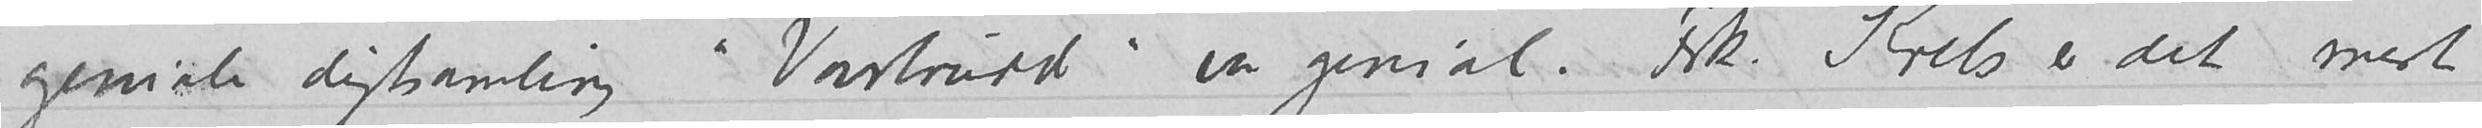geniale digtsamling «Vaarbrudd» var genial.  Frk. Krebs er det mest
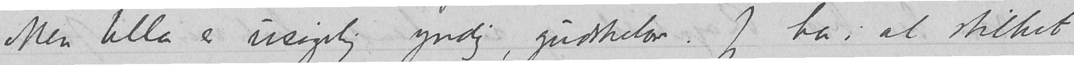Men Ulla er usigelig yndig, gudskelov.  Jeg har i al stilhet
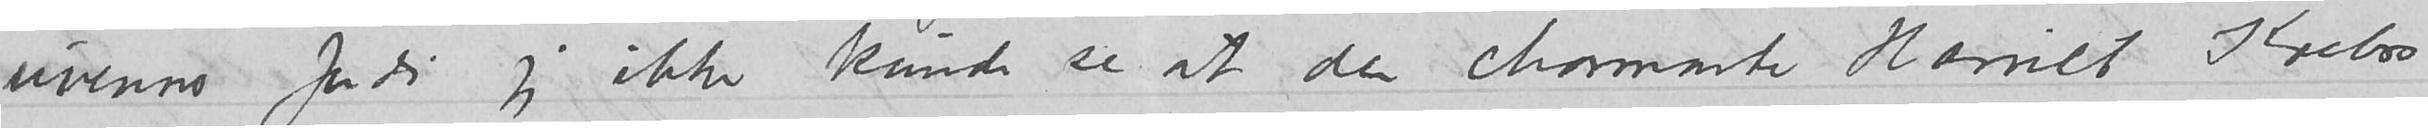uvenner fordi jeg ikke kunde se at den charmante Harriet Krebss
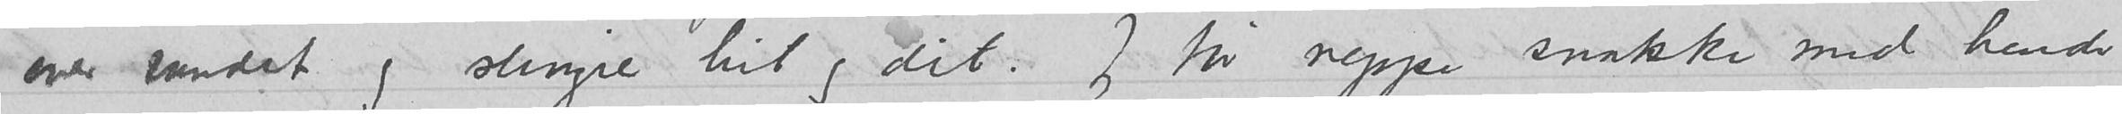over vandet og slingrer hit og dit.  Jeg får neppe snakke med hende
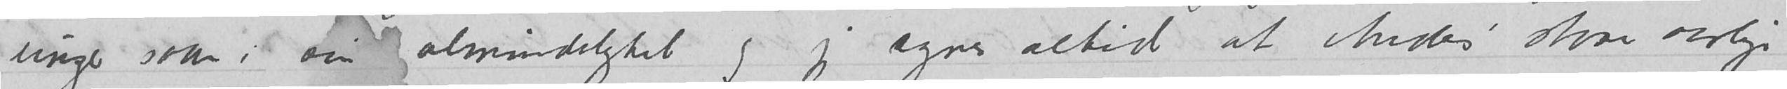unger saan i sin almindelighet og jeg synes altid at Anders’ store aarlige
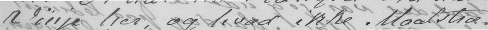Vinje her, og hvad ikke Maalstræ-
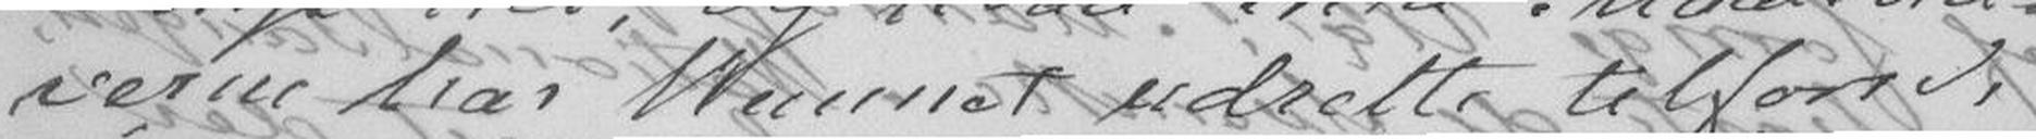verne har kunnet udrette tilforn,
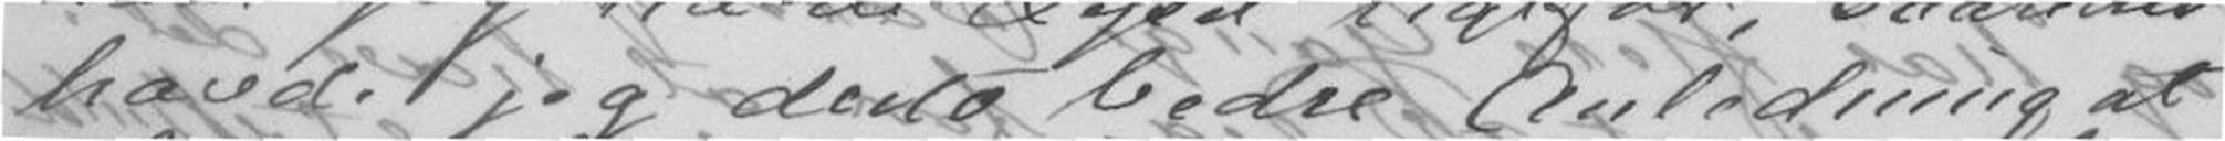havde jeg desto bedre Anledning at
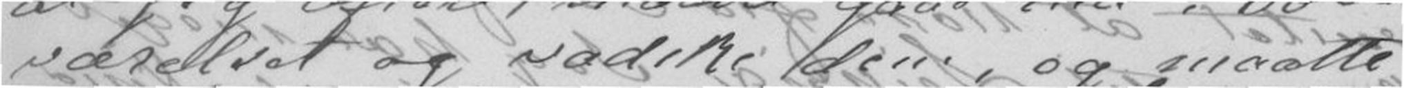værelset og vadske dem, og maatte
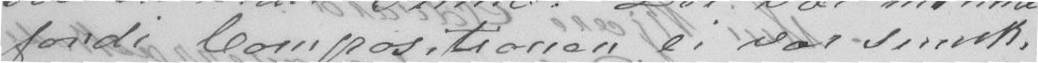fordi Compositionen ei var smuk,
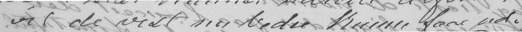vil de vist nu bedre kunne faae ud-
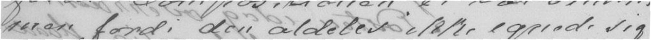men fordi den aldeles ikke egnede sig
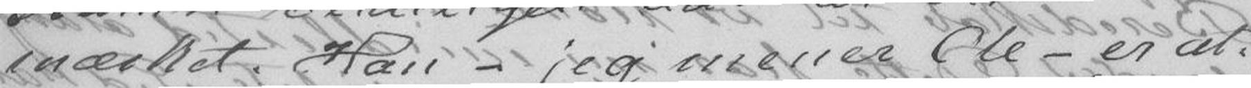mærket. Han - jeg mener Ole - er at-
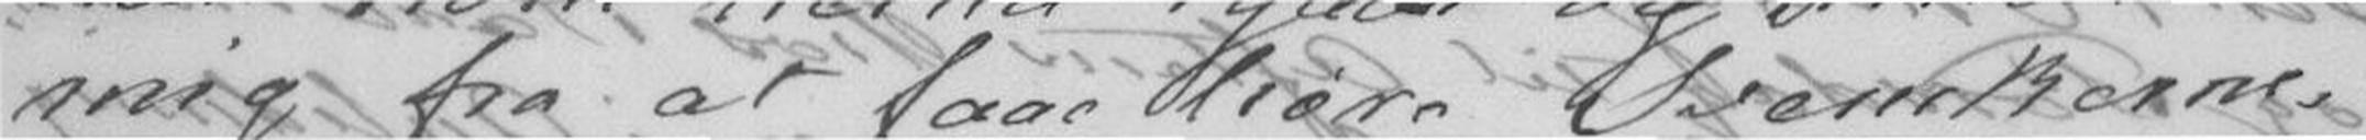mig fra at faae høre Svenskerne,
